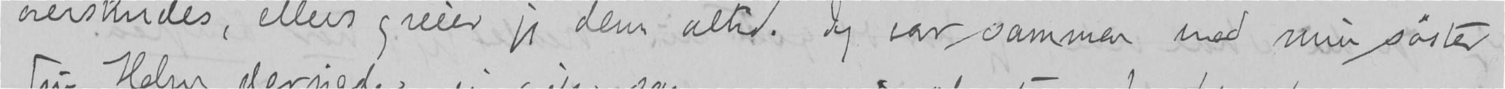overskrides, ellers greier jeg dem altid. Jeg var sammen med min søster
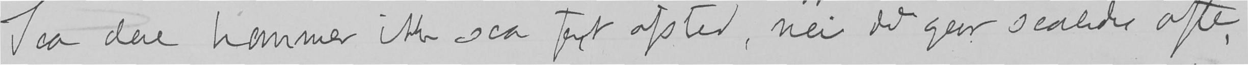Saa dere kommer ikke saa fort afsted, nei det gaar saaledes ofte,
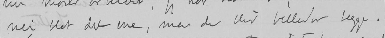nei blot det ene, men de blir billeder begge.
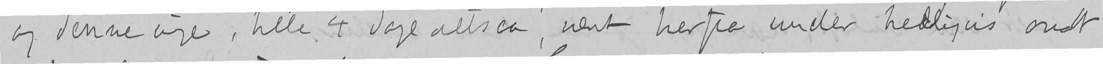og denne uge, hele 4 dage altsaa, været herfra under heldigvis ondt
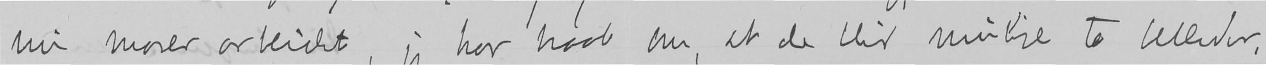nu morer arbeidet, jeg har haab om, at de blir mulige to billeder,
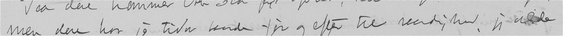men dere har jo tiden baade før og efter til raadighed, jeg vilde
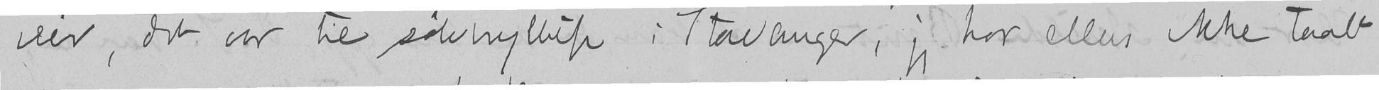veir, det var til sølvbryllup i Stavanger, jeg har ellers ikke taalt
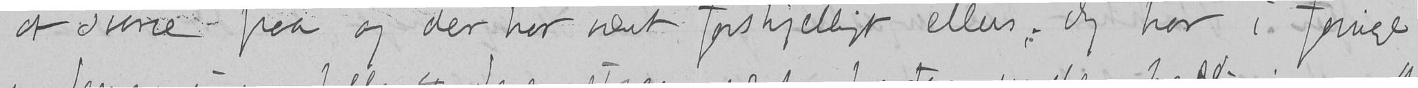at svare paa og der har været forskjelligt ellers. Jeg har i forrige
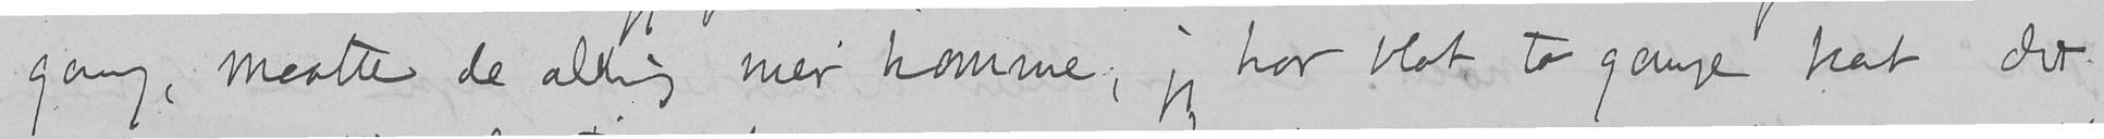gang, maatte de aldrig mer komme, jeg har blot to gange hat det
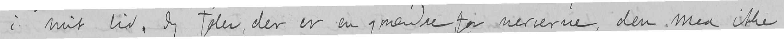i mit liv. Jeg føler, der er en grændse for nerverne, den maa ikke
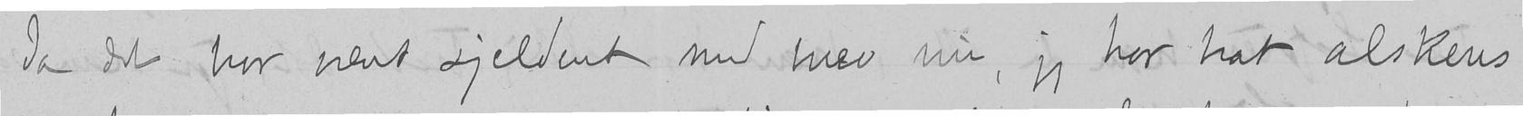Ja det har været sjeldent med brev nu, jeg har hat alskens
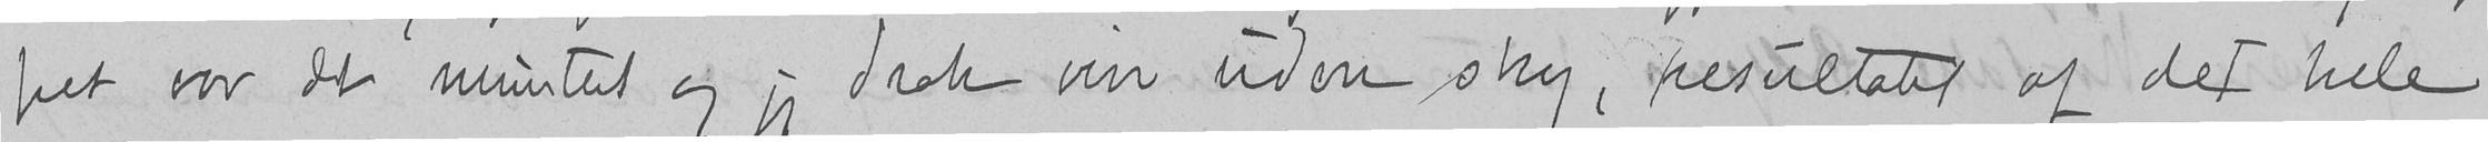pet var det muntert og jeg drak vin uden sky, resultatet af det hele
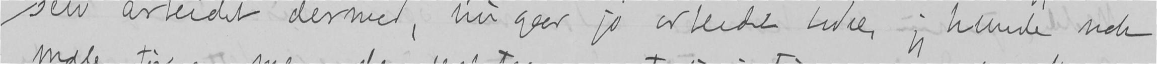selv arbeidet dermed, nu gaar jo arbeidet bedre, jeg kunde nok
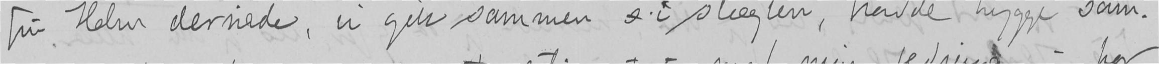fru Holm dernede, vi gik sammen s i slægten, havde hygge sam-
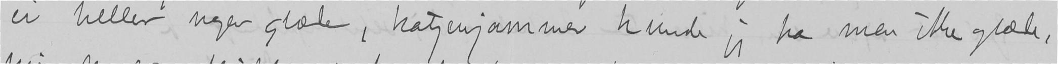ei heller nogen glæde, katzenjammer kunde jeg ha men ikke glæde,
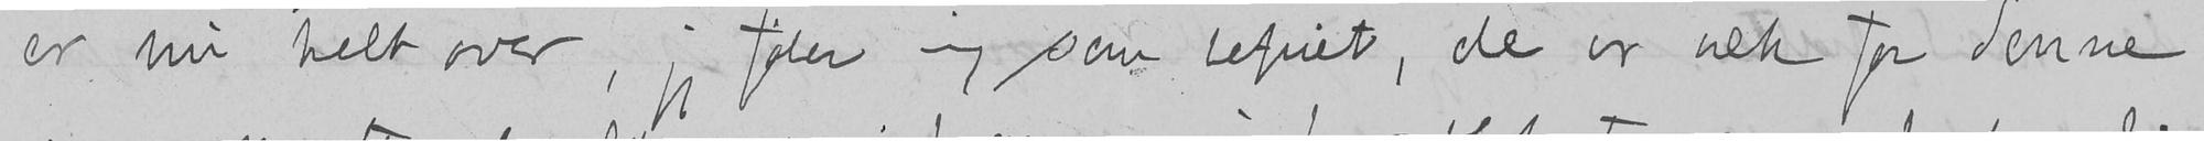er nu helt over, jeg føler mig som befriet, de er væk for denne
